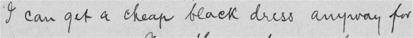I can get a cheap black dress anyway for
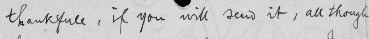thankfull, if you will send it, all though
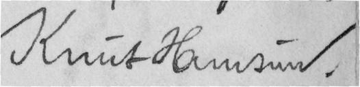Knut Hamsun.
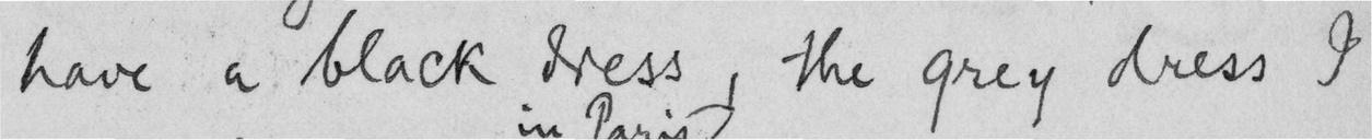have a black dress, the grey dress I
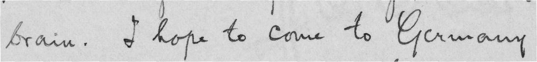brain. I hope to come to Germany
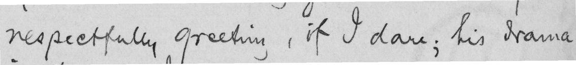respectfully greeting, if I dare; his drama
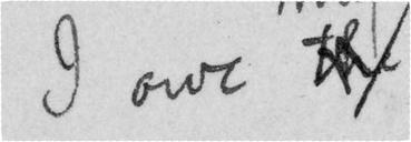I owe the
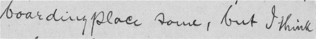boarding place some, but I think
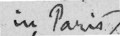in Paris
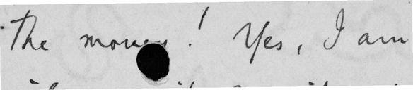The money! Yes, I am
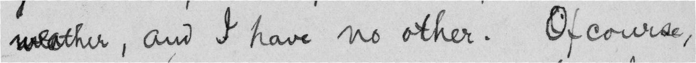weather, and I have no other. Of course,
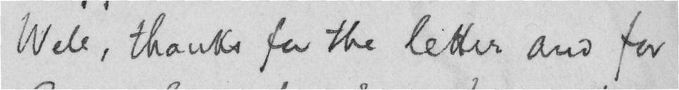Well, thanks for the letter and for
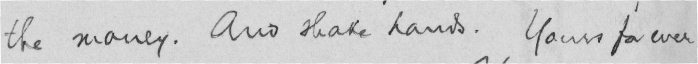the money. And shake hands. Yours for ever
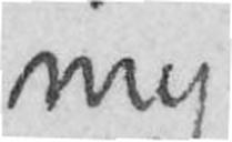my
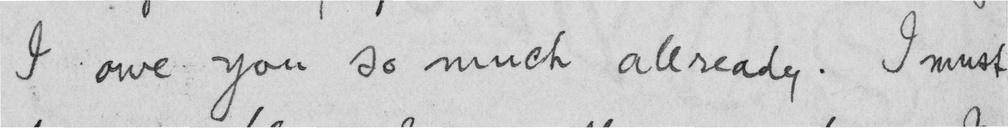I owe you so much allready. I must
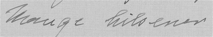Mange hilsener
